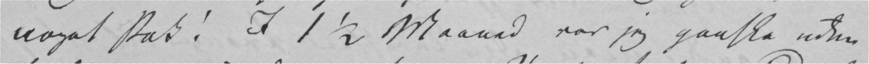noget Pak! I 1 ½ Maaned var jeg ganske uden
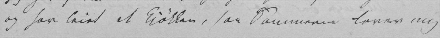og har leiet et Kjøkken, saa Sommeren lover mig
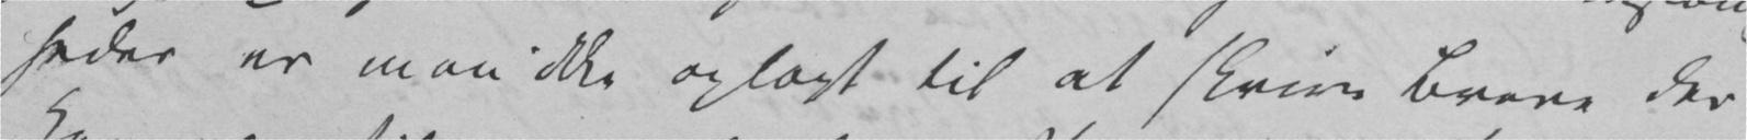er man ikke oplagt til at skrive Breve der
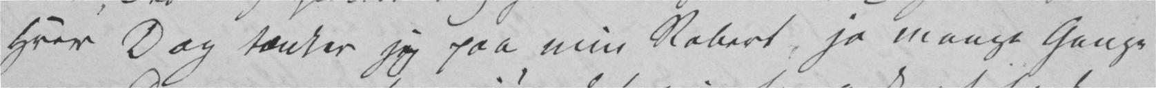Hver Dag tænker jeg paa min Robert, ja mange Gange
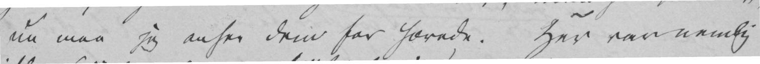nu maa jeg ansee dem for hævede. Her var nemlig
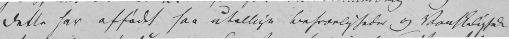dette har affødt saa utallige Besværligheder og Vanskeligheder
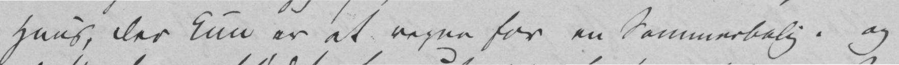Huus, der kun er at regne for en Sommerbolig. og
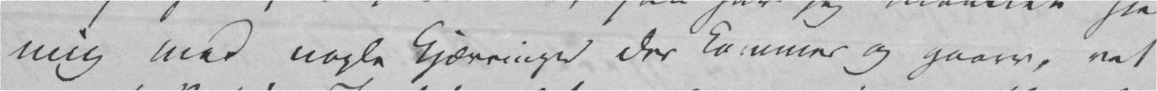mig med nogle Kjærringer der kommer og gaaer, ret
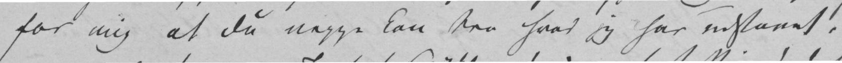for mig at Du neppe kan tro hvad jeg har udstaaet i
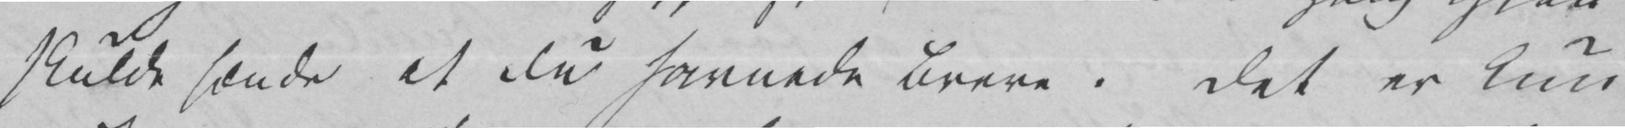skulde hænde at Du savnede Breve. Det er kun
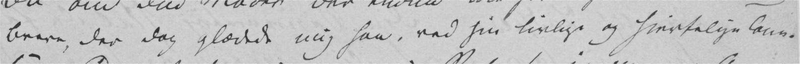Breve, der dog glædede mig saa, ved sin livlige og hjertelige Tone.
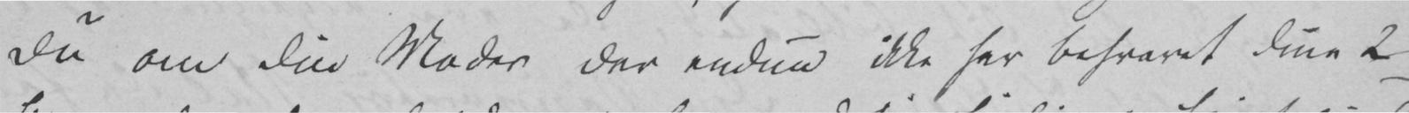Du om Din Moder der endnu ikke har besvaret Dine 2
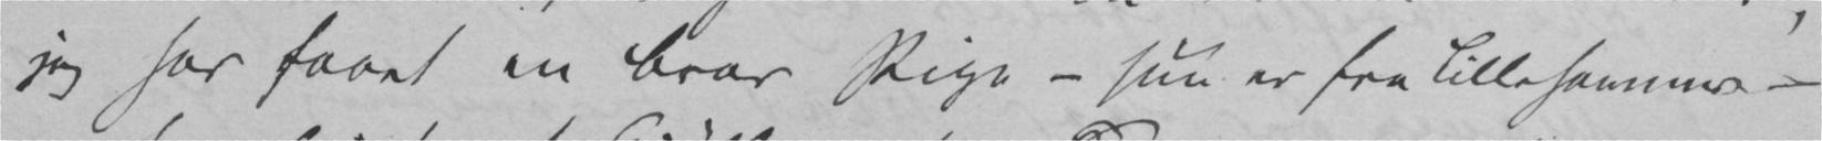jeg har faaet en brav Pige – hun er fra Lillehammer –
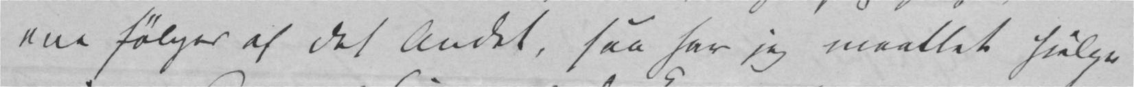ene følger af det Andet, saa har jeg maattet hjelpe
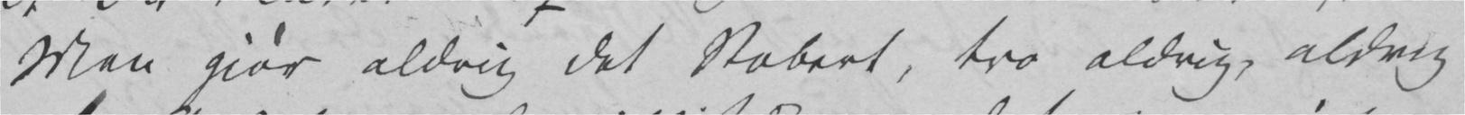Men gjør aldrig det Robert, tro aldrig, aldrig
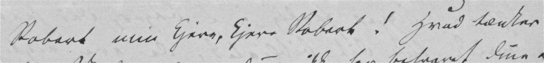Robert min kjere, kjere Robert! Hvad tænker
